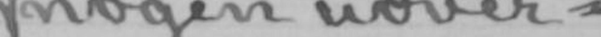nogen uover-
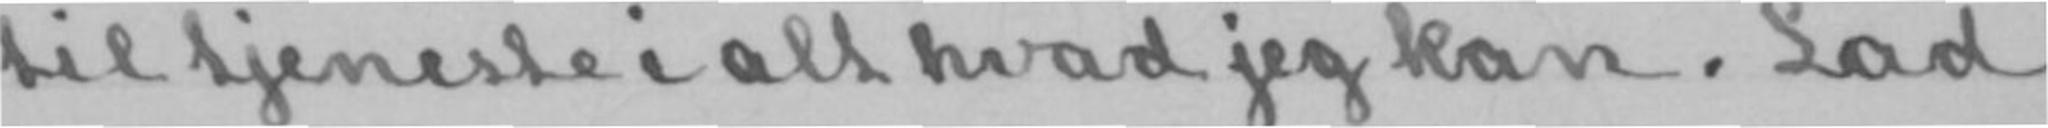til tjeneste i alt hvad jeg kan. Lad
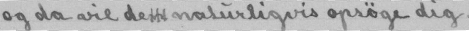og da vil den naturligvis opsøge dig.
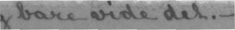mig bare vide det.-
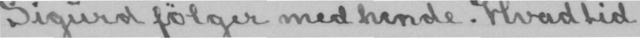Sigurd følger med hende. Hvad tid,
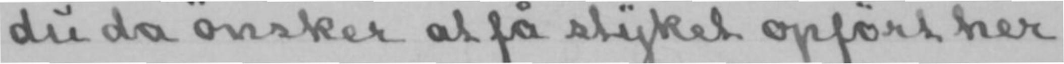du da ønsker at få styket opført her,
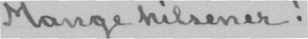Mange hilsener!
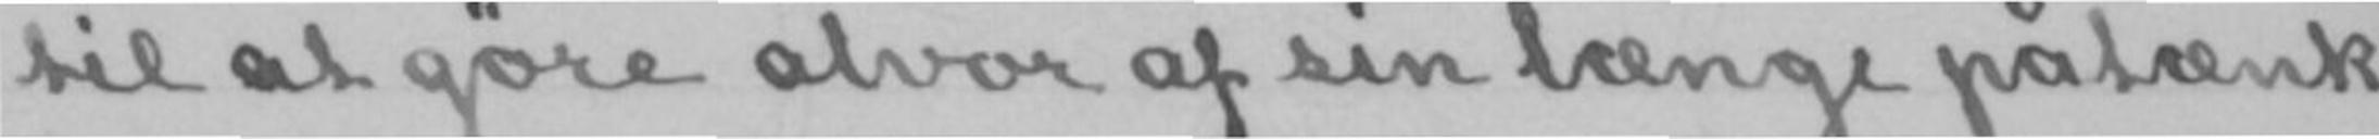til at gøre alvor af sin længe påtænk-
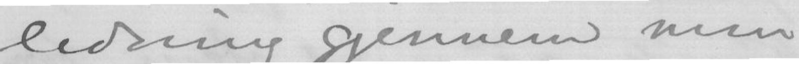ledning gjennem min
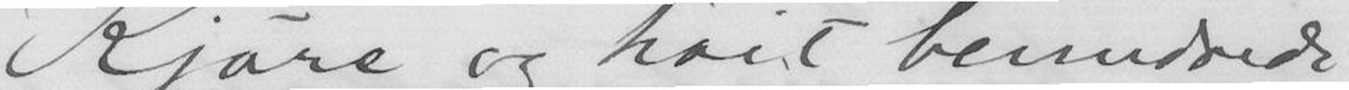Kjære og høit beundrede
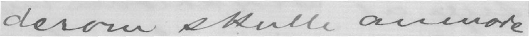derom skulle anmode
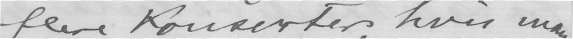flere Konserter, hvis man
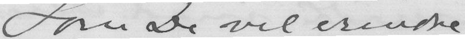Som De vil erindre
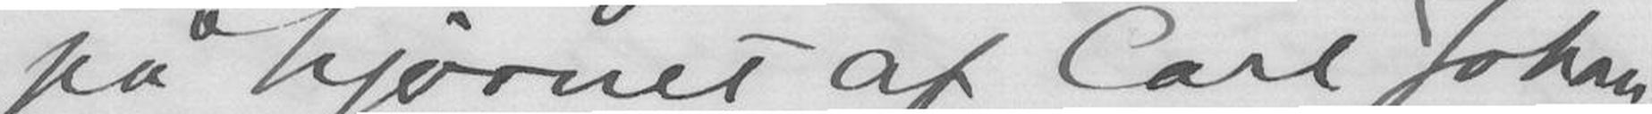på hjørnet af Carl Johan
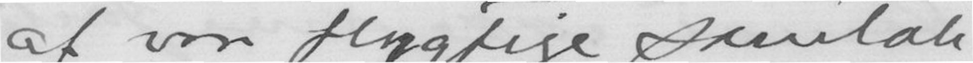af vor flygtige samtale
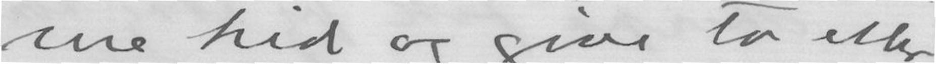me hid og give to eller
